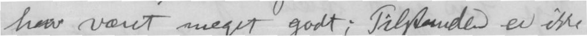har været meget godt; Tilstanden er ikke
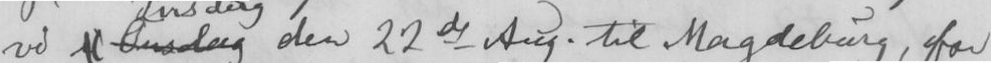vi i Onsdag Tirsdag den 22de Aug. til Magdeburg, for
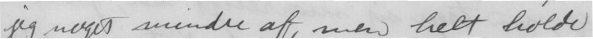jeg noget mindre af, men helt holde
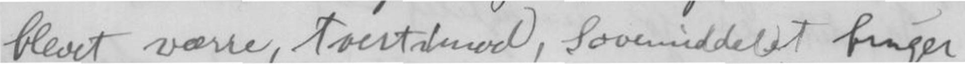blevet værre, tvertimod, Sovemiddelet trenger
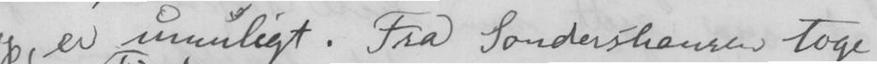op, er umuligt. Fra Sondershausen toge
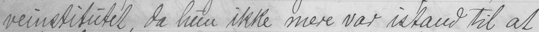veinstitutet, da hun ikke mere var istand til at
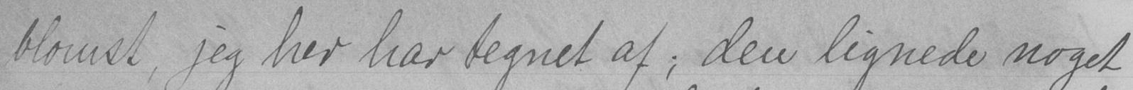blomst, jeg her har tegnet af; den lignede noget
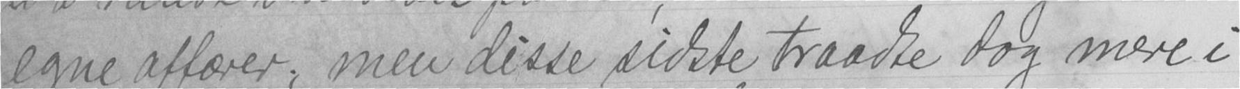egne affærer; men disse sidste traadte dog mere i
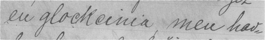en glockcinia, men hav-
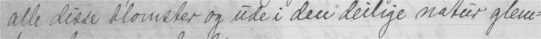alle disse blomster og ude i den deilige natur glem-
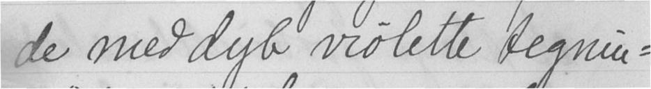de med dyb violette tegnin-
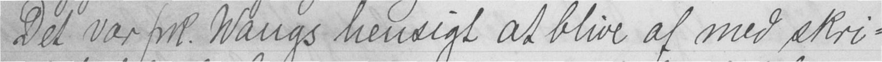Det var frk. Wangs hensigt at blive af med skri-
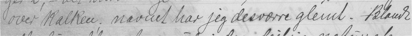over kalken; navnet har jeg desværre glemt. Blandt
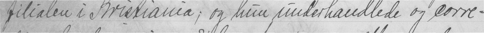filialen i Kristiana; og hun underhandlede og corre-
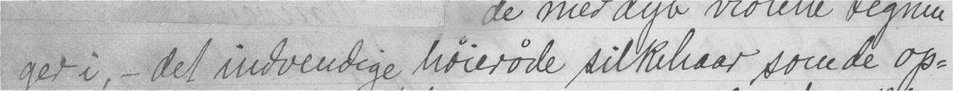ger i, - det indvendige høierøde silkehaar som de op-
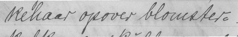kehaar opover blomster-
